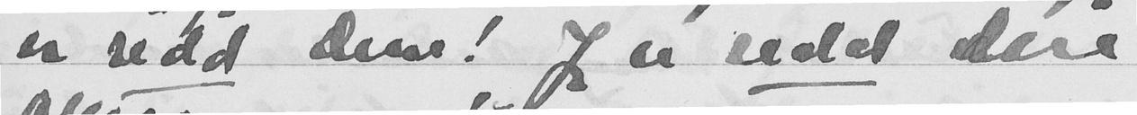er redd Dem! Jeg er redd dere
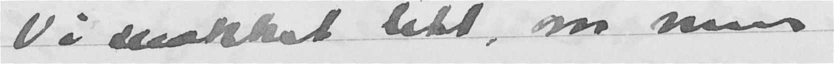Vi snakket litt, om min
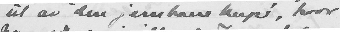ut av den jernbanekupé, hvor
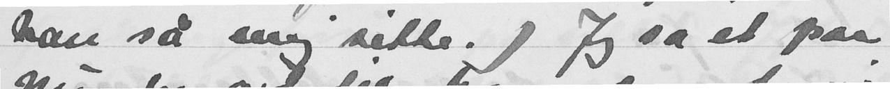han så mig sitte.) Jeg sa et par
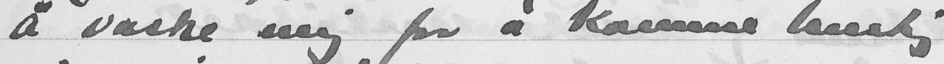å vaske mig for å komme hurtig
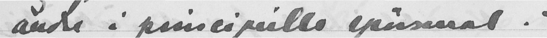andre i principielle spørsmål."
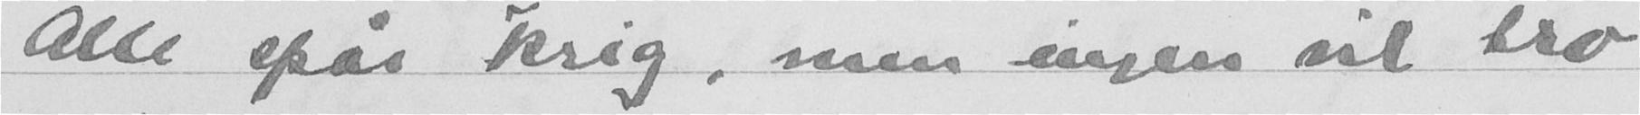Alle spår krig, men ingen vil tro
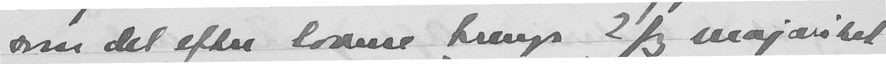som det efter lovene trenger 2/3 majoritet
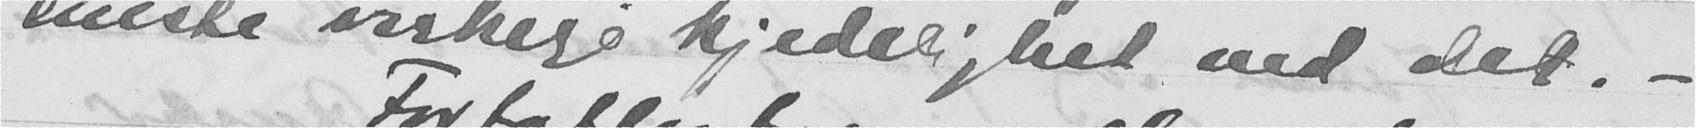eneste virkelige kjedelighet ved det. -
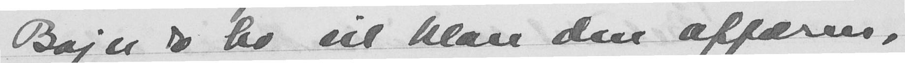Bojer & co vil klare den affæren,
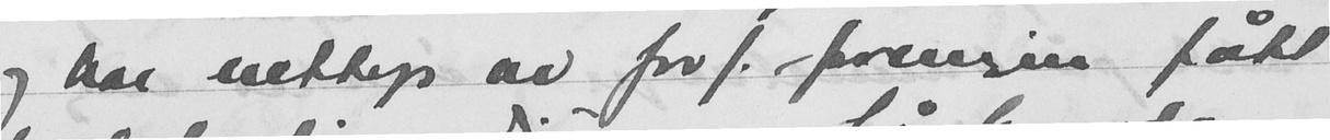og har nettop av forf. foreningen fått
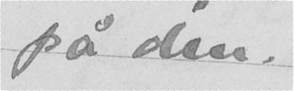på den.
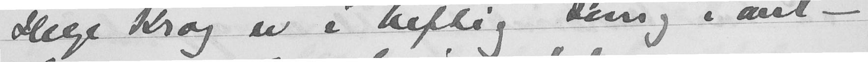Helge Krog er i heftig sving i anl -
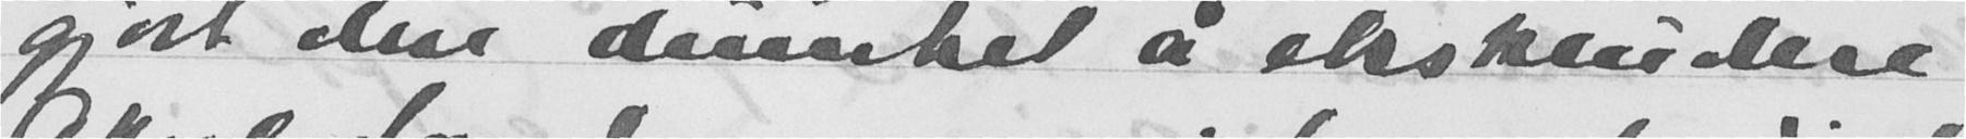gjort den dumhet å ekskludere
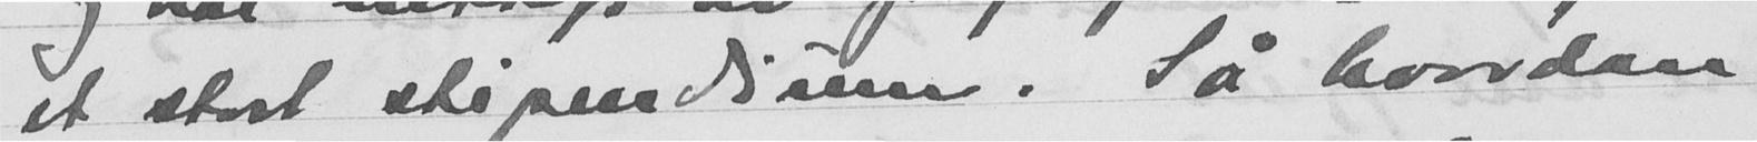et stort stipendium. Så hvordan
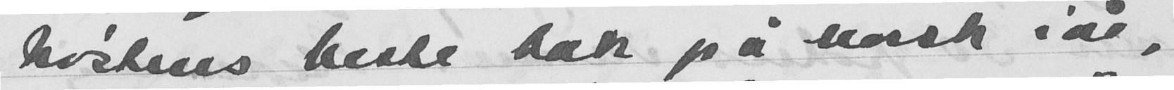høstens beste bok på norsk iår,
